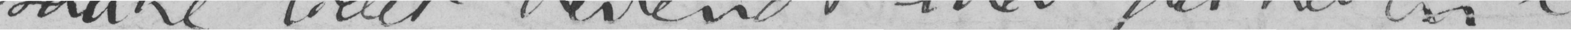saare lidet bevendt med friheden i
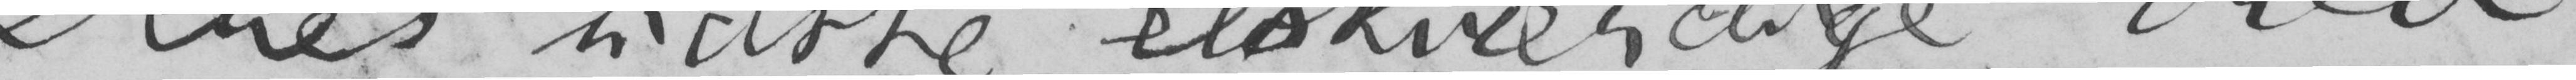Deres sidste elskværdige brev!
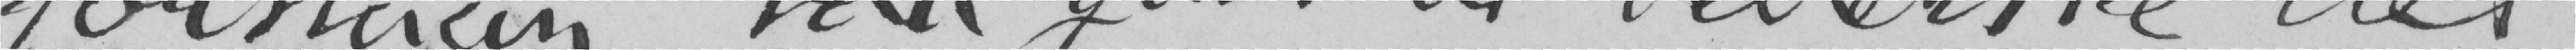forstaar saa godt at beherske det
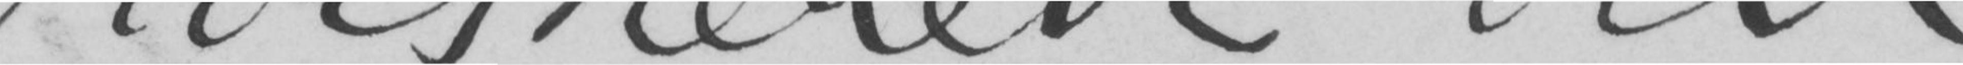Høistærede ven!
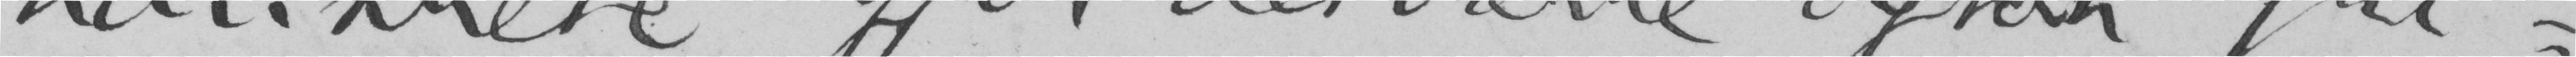konkrete, gjør dessværre ogsaa fri-
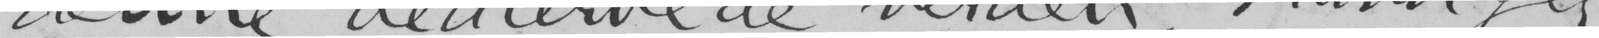denne bedærvede verden. Havde jeg
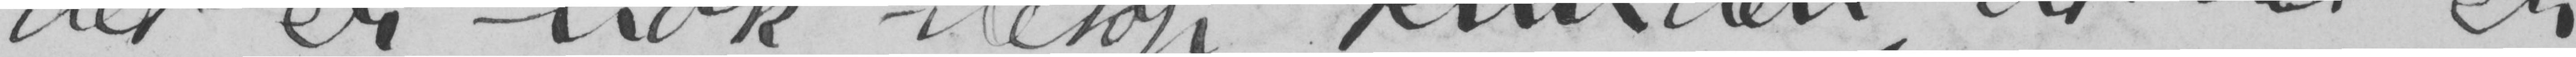det er nok netop knuden, at det er
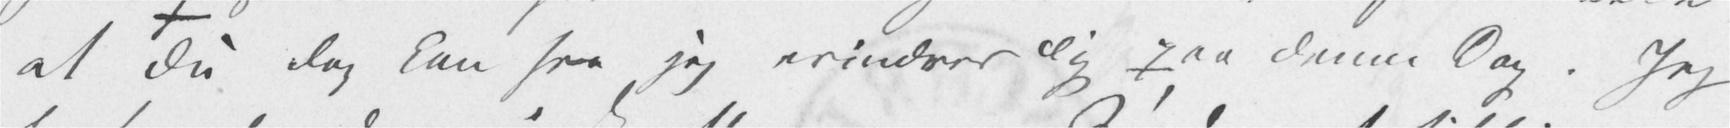at Du dog kan see jeg erindrer Dig paa denne Dag. Jeg
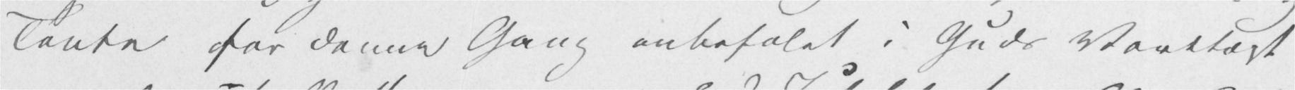Tante for denne Gang anbefalet i Guds Varetægt
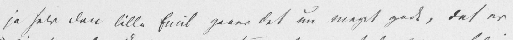ja selv den lille Emil gaaer det nu meget godt, det er
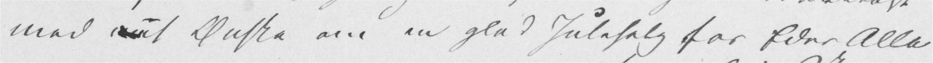med miet Ønske om en glad Julehelg for Eder Alle
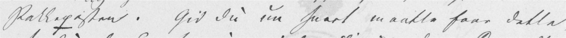Pakkeposten. Gid Du nu snart maatte faae dette,
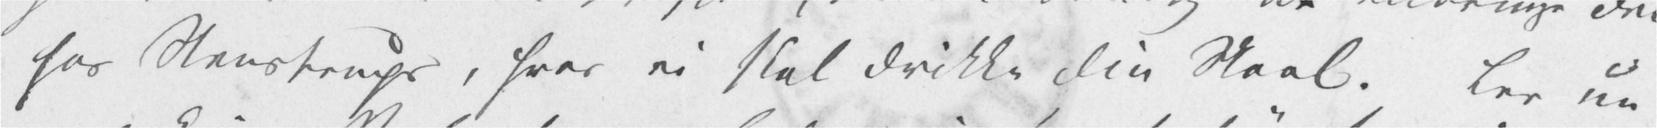hos Stenstrups, hvor vi skal drikke Din Skaal. Lev nu
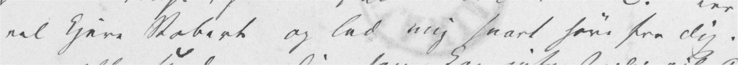vel kjere Robert og lad mig snart høre fra Dig.
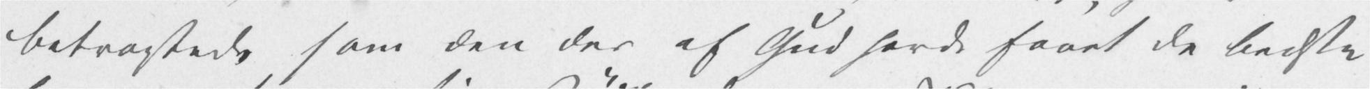betragtede som den der af Gud havde faaet de bedste
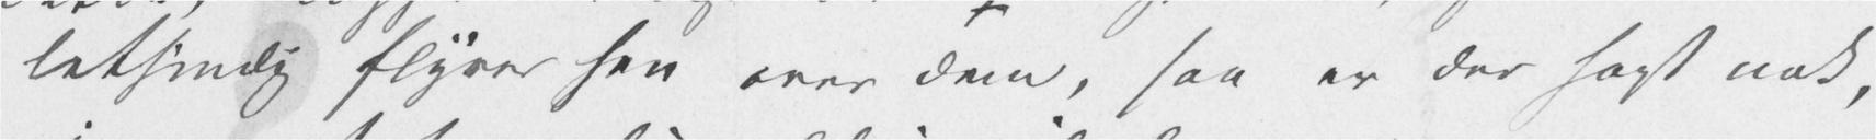letsindig flyver hen over dem, saa er der sagt nok,
